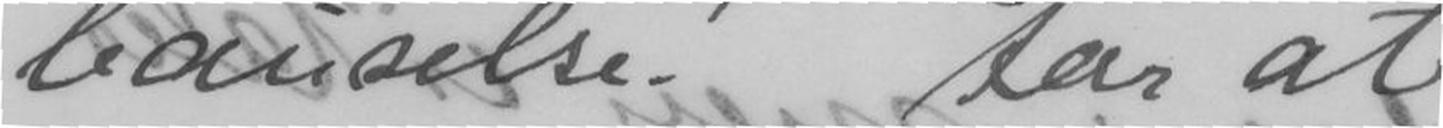bauselse! For at
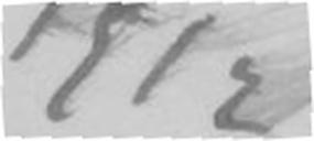1912
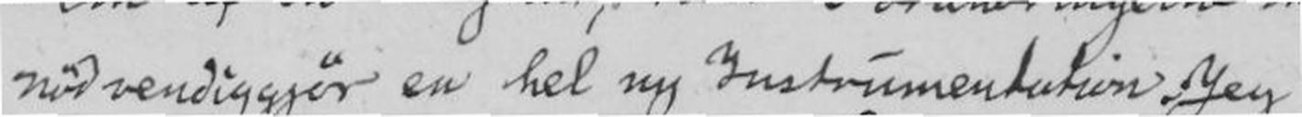nødvendiggjør en hel ny Instrumentation. Jeg
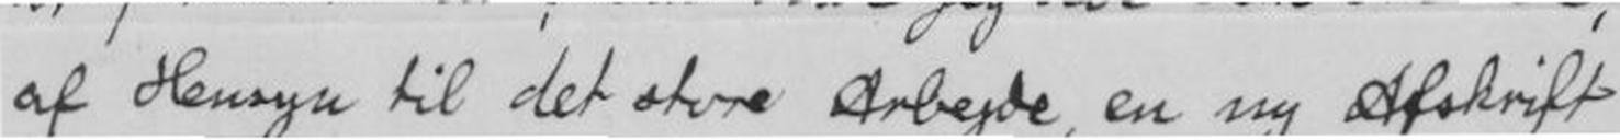af Hensyn til det store Arbeide, en ny Afskrift
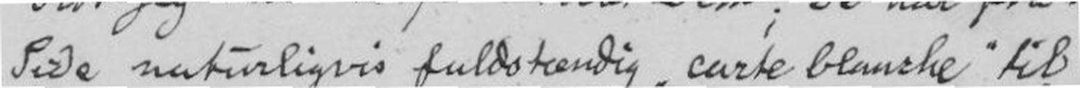Side naturligvis fulstændig "Carte blanche" til
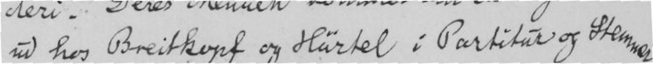ud hos Breitkopf og Hartel i Partitur og Stemmer
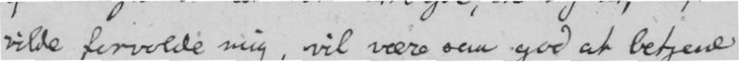vilde forvolde mig, vil være saa god at betjene
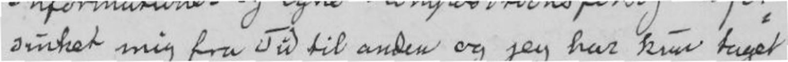untret mig fra Tid til anden og jeg har kun taget
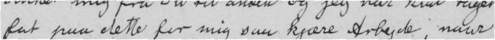fat paa dette for mig saa kjære storbyde, naar
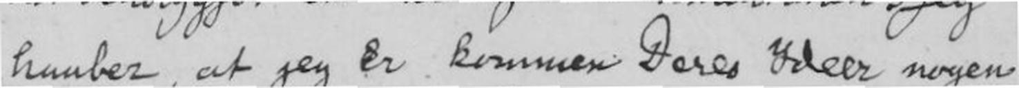haaber, at jeg er kommen Deres Ideer nogen
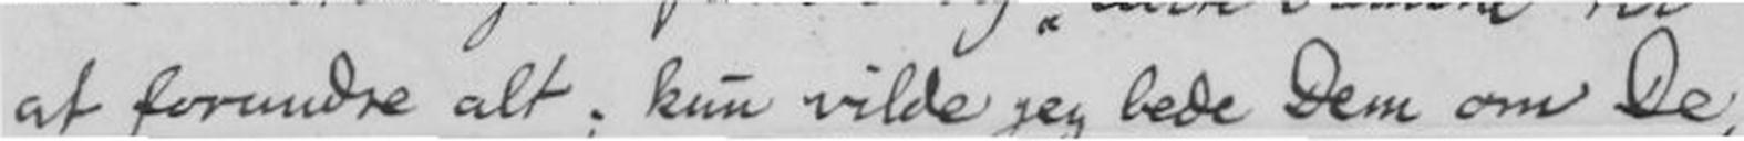at forundre alt; kun vilde jeg bede Dem om De,
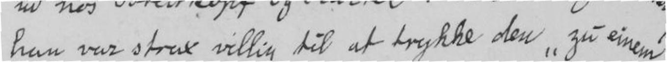han var strax villig til at trykke den "zu eimem
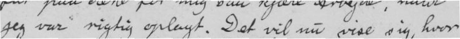jeg var rigtig oplagt. Det vil nu vise sig, hvor
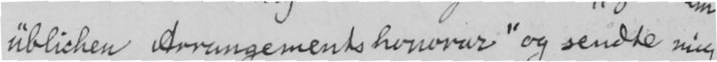üblichen Arrangements honorar" og sendte mig
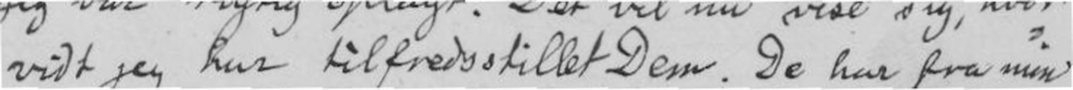vidt jeg har tilfredsstillet Dem; De har fra min
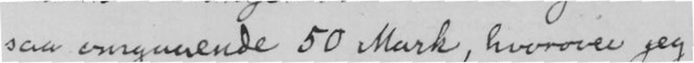saa omgaaende 50 mark, hvorover jeg
